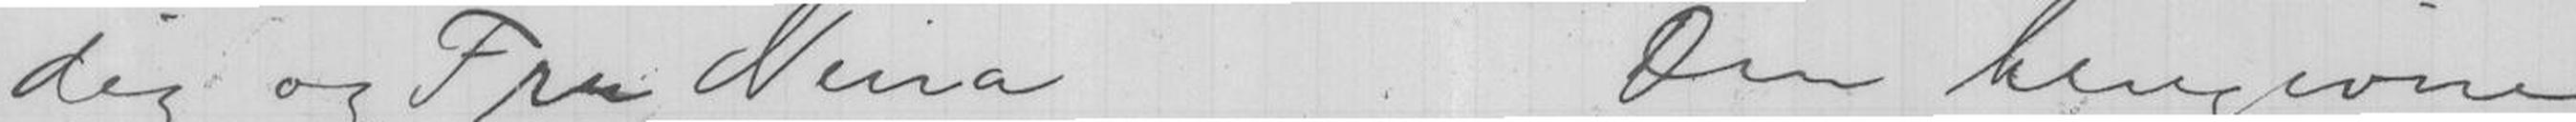dig og Fru Nina Din hengivne
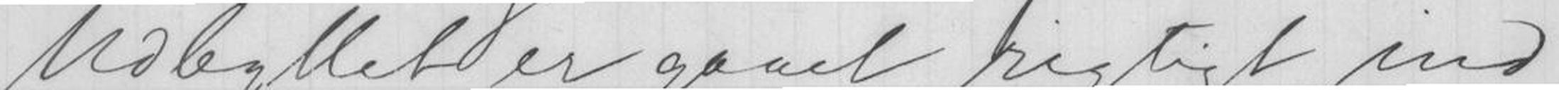Udbyttet er gaaet rigtigt ind
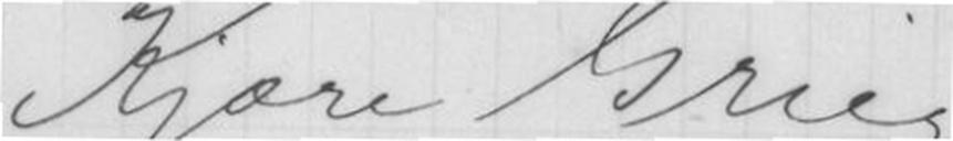Kjære Grieg!
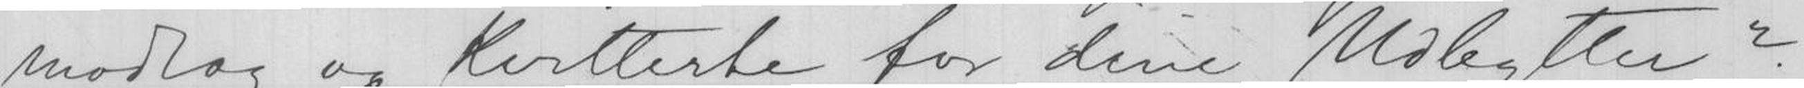modtog og Kvitterte for dine Udbytter?
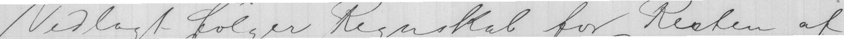Vedlagt følger Regnskab for Resten af
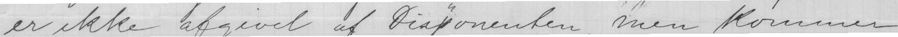er ikke afgivet af Disponenten, men kommer
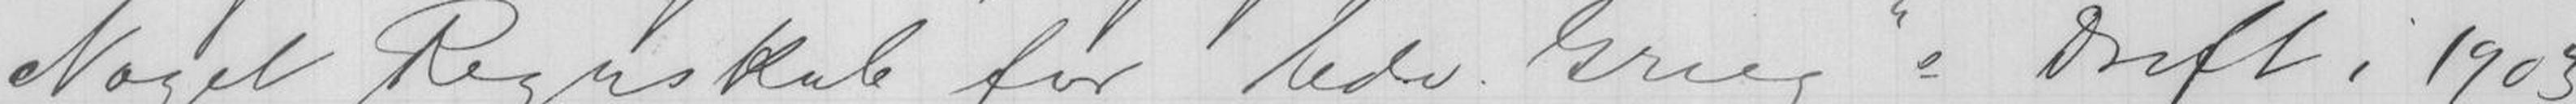Noget Regnskab for Edv. Griegs Drift i 1903
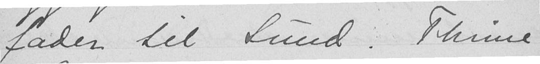fader til Sund. Thrine
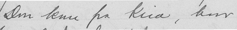Don kom fra Kria, hvor
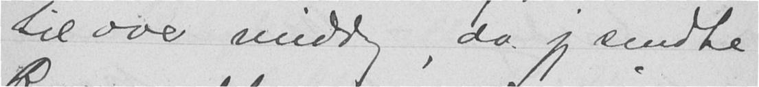til over middag da jeg sendte
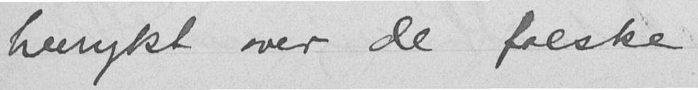henrykt over de falske
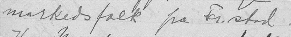markedsfolk fra Fr.stad.
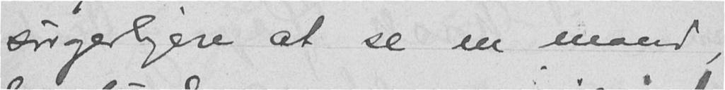sørgeligere at se en mand,
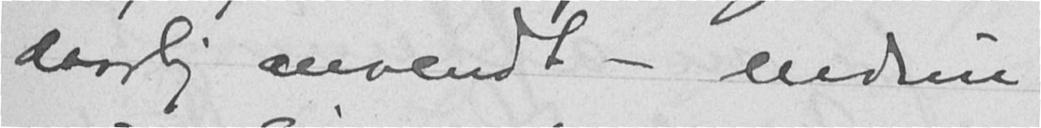daarlig anvendt - endnu
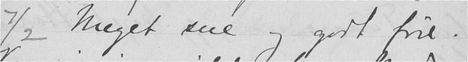7/2 Meget sne og godt føre.
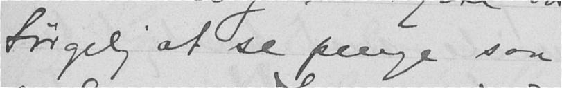Sørgelig at se penge saa
